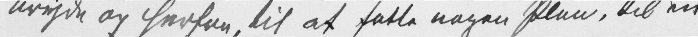bryde op herfra, til at fatte nogen Plan, til en
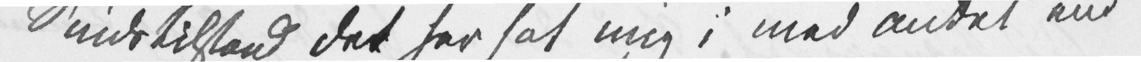Sindstilstand det har sat mig i med andet end
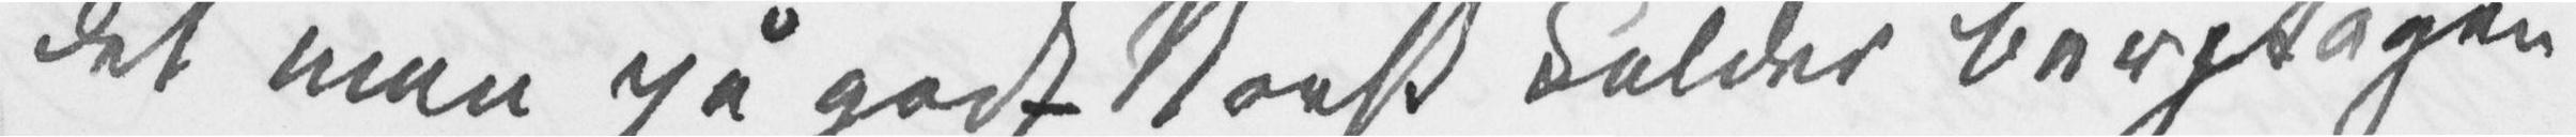det man på godt Norsk kalder «berjtagen»
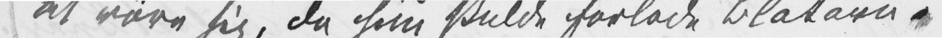at røre sig, da hun skulde forlade Blåtårn.
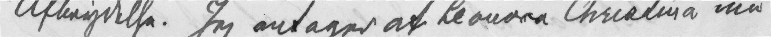Afbrydelse. Jeg antager at Leonora Christina må
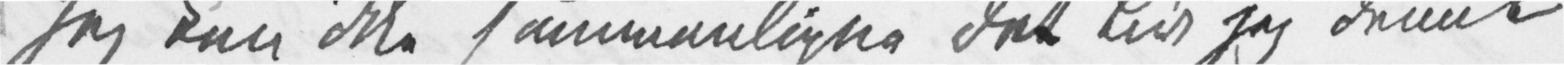Jeg kan ikke sammenligne det Liv jeg denne
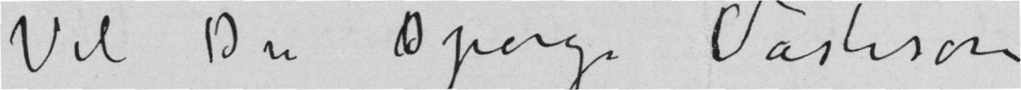Vil du spørge Vasteson
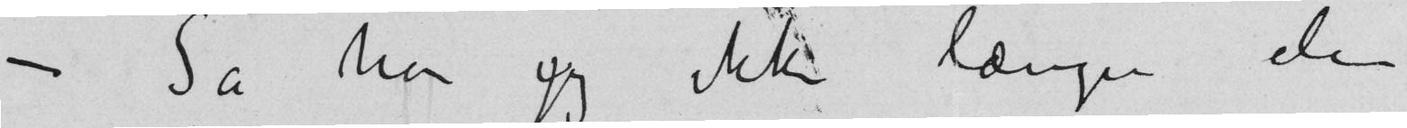Så har jeg ikke lenger den
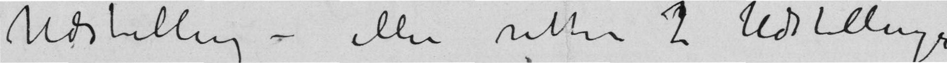Udstilling – eller rettere 2 Udstillinger
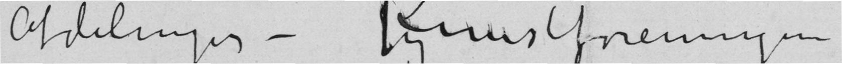Afdelinger – Kunstforeningen
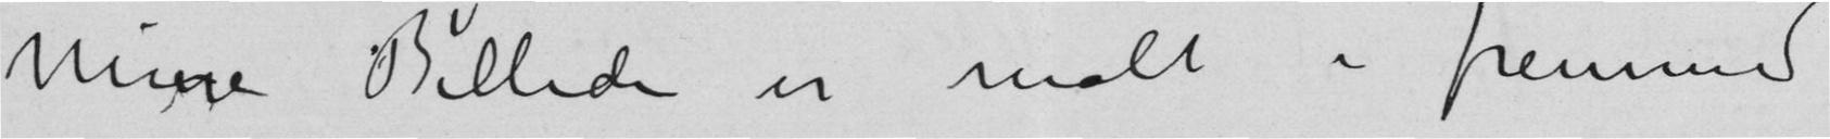Mine Billeder er malt i fremmed
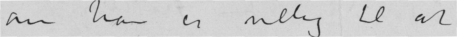om han er villig til at
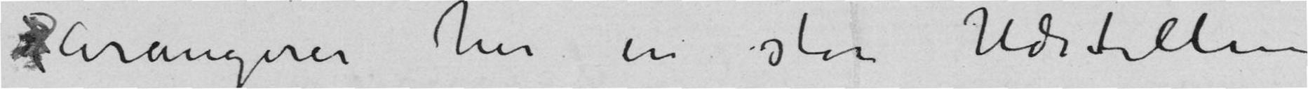arangerer her en stor Udstilling
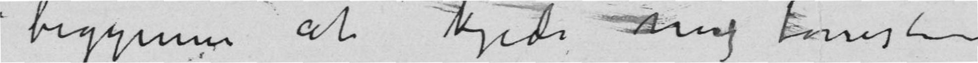begynner at kjede mig forresten
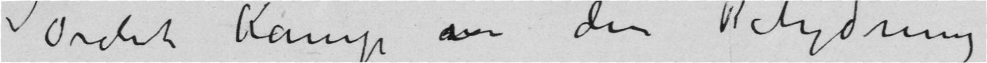*)ordet Kamp i den Betydning
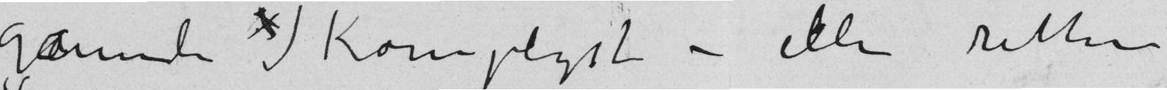gamle *) Kamplyst – eller rettere
